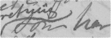som har
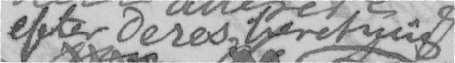efter Deres beretning
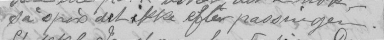så spørs det ikke efter passingen.
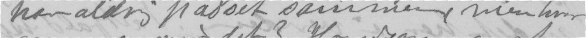har aldrig passet sammen, men hvor
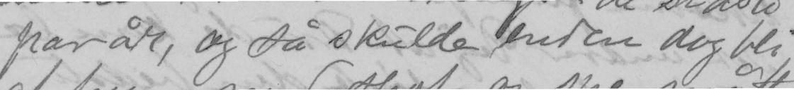par år, og så skulde enden dog bli
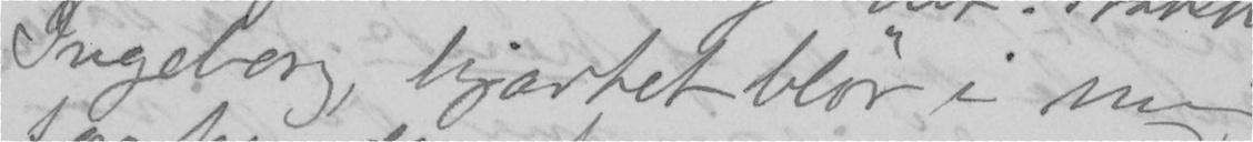Ingeborg, hjærtet blør i mig
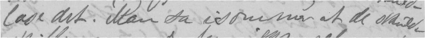læse det. Man sa isommer at de skulde
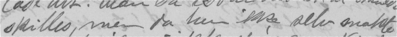skilles, men da hun ikke selv snakte
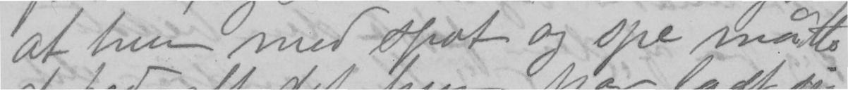at hun med spot og spe måtte
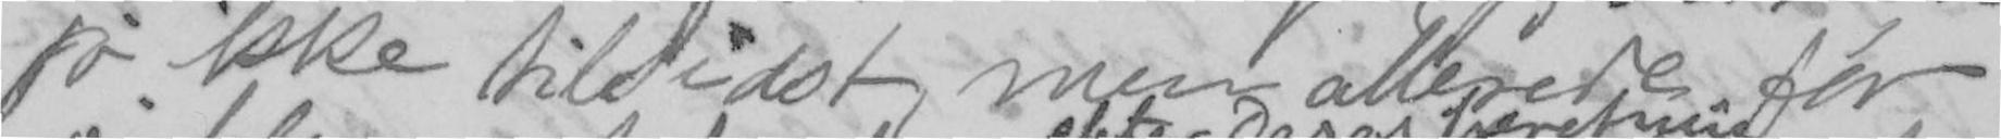jo ikke tilsidst, men allerede før
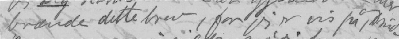brænde dette brev, for jeg er vis på, Drude
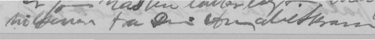hilsener fra Din Amalie Skram
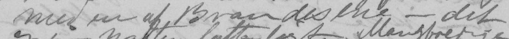med en af Brandesene - det
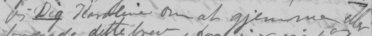jeg Dig Karoline om at gjemme eller
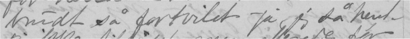brudt så fortvilet -ja, jeg så hende
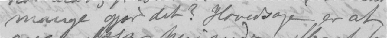mange gør det? Hovedsagen er at
